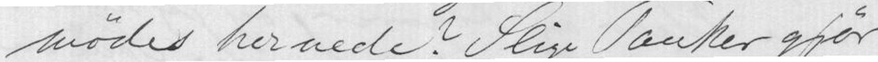mødes her nede? Slige Tanker gjør
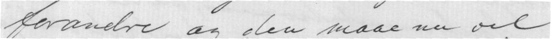forandre og den maae nu vel
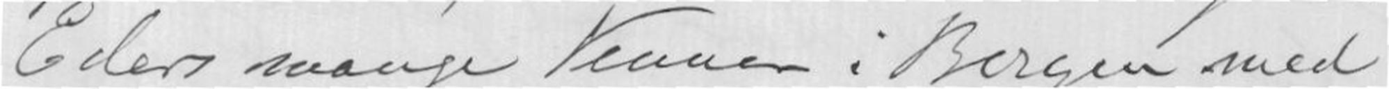Eders mange Venner i Bergen med
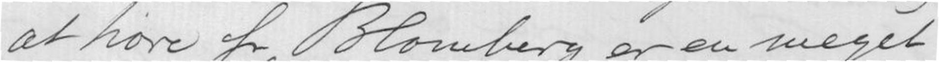at høre hr Blomberg er en meget
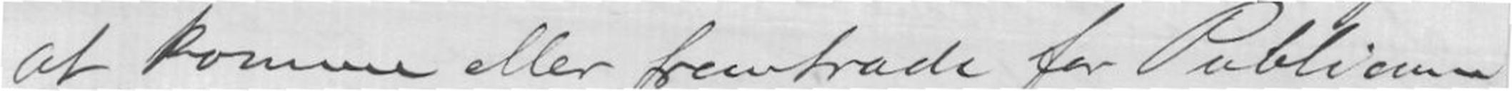at komme eller fremtræde for Publicum
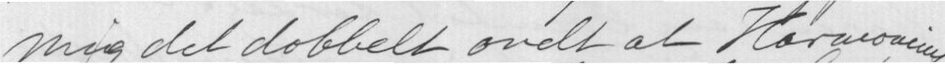mig det dobbelt ondt at Harmoniens
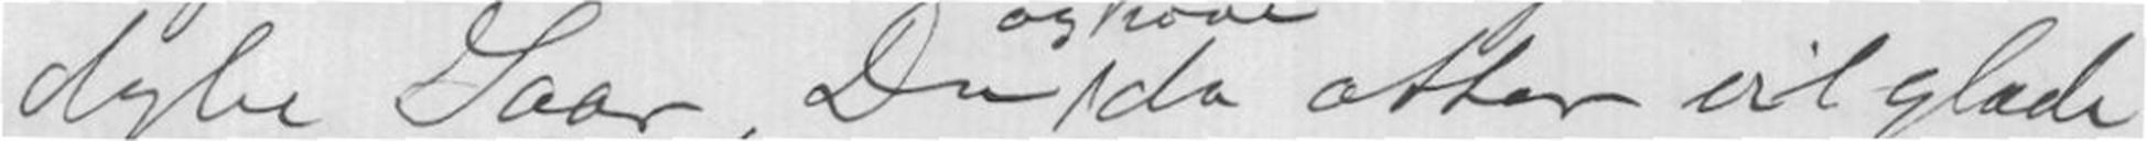dybe Saar, Du da atter vil glæde
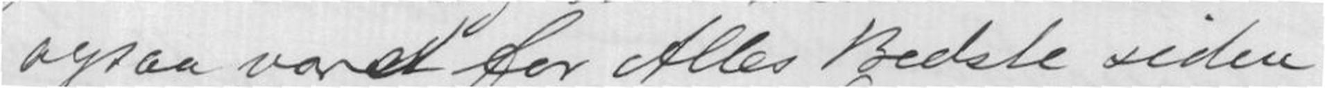ogsaa været for Alles Bedste siden
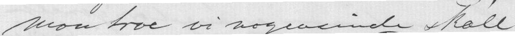mon troe vi nogensinde skall
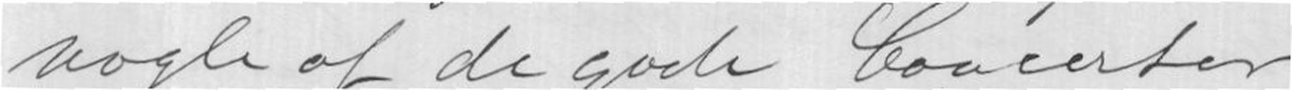nogle af de gode Concerter
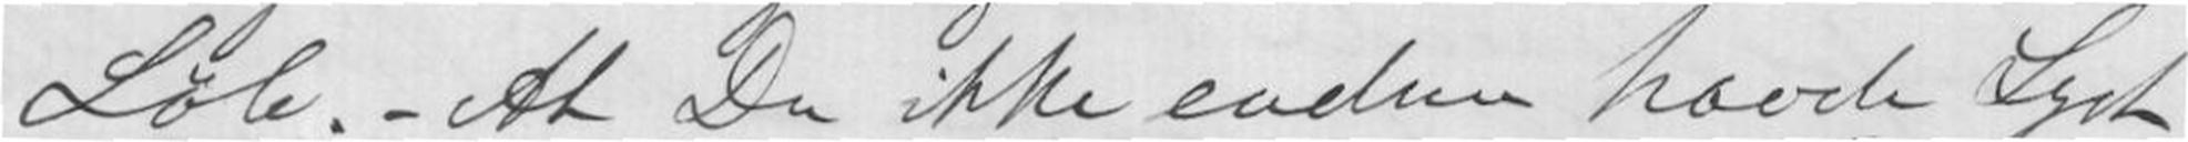Løb. - At Du ikke endnu havde Lyst
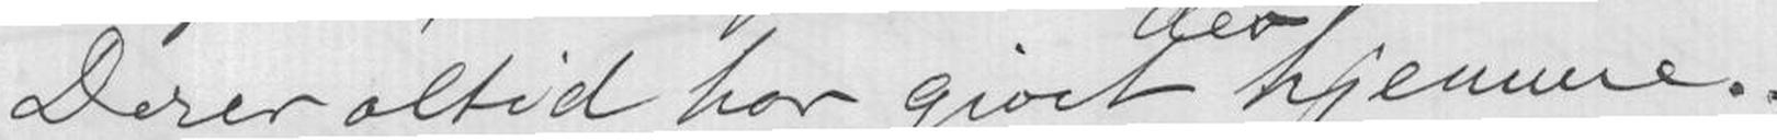Derer altid har givet hjemme. -
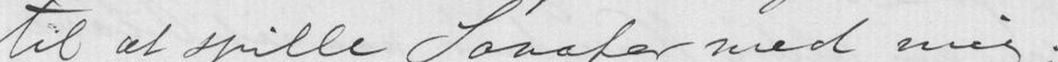til at spille Sonater med mig;
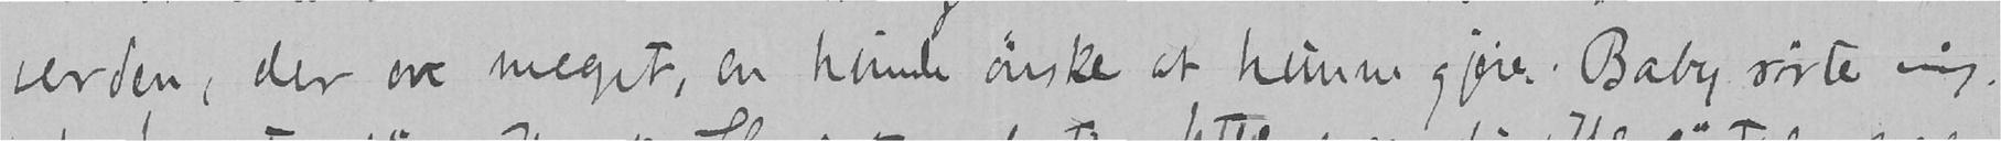verden, der er meget, en kunde ønske at kunne gjøre. Baby rørte mig.
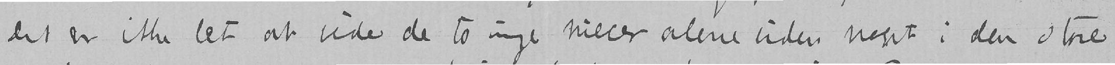Det er ikke let at vide de to unge niecer alene uden noget i den store
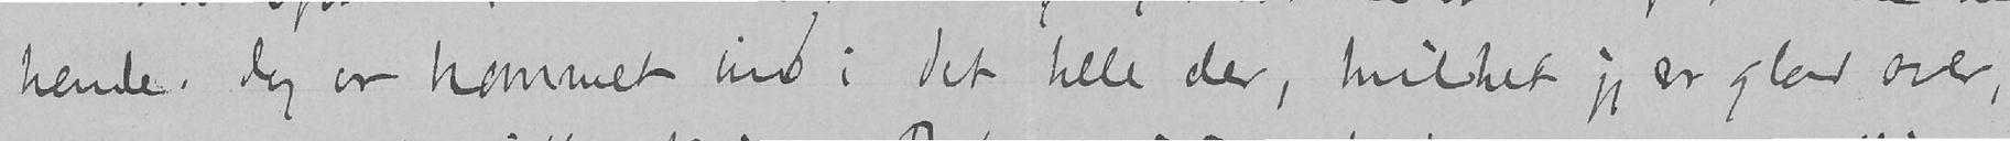hende. Jeg er kommet ind i det hele der, hvilket jeg er glad over,
